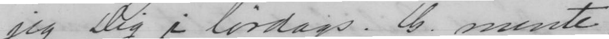jeg Dig i lørdags. G. mente,
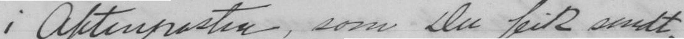i Aftenposten, som Du fik sendt.
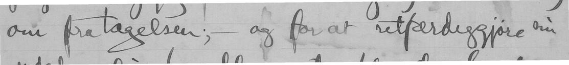om fratagelsen; - og for at retfærdiggjøre sin
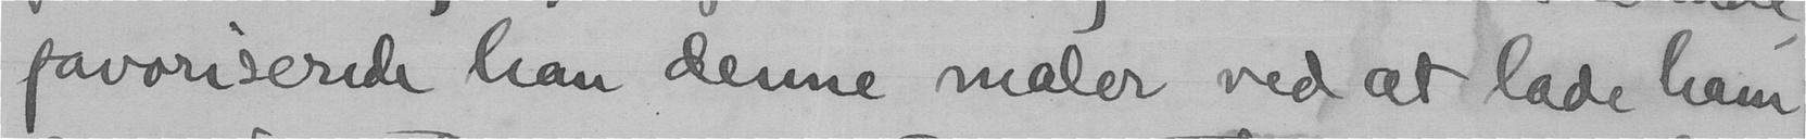favoriserede han denne maler ved at lade ham
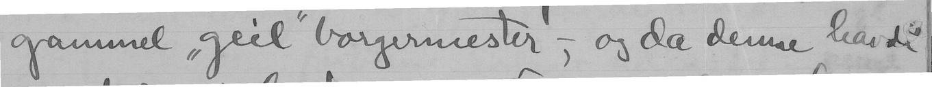gammel "geil" borgermester, - og da denne havde
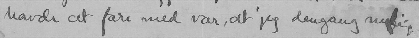havde at fare med var, at jeg dengang nylig
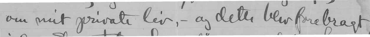om mit private liv, - og dette blev forebragt
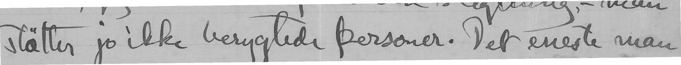støtter jo ikke berygtede personer. Det eneste man
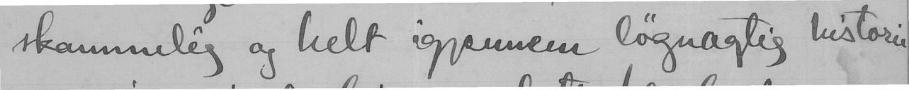skammelig og helt igjennom løgnagtig historie
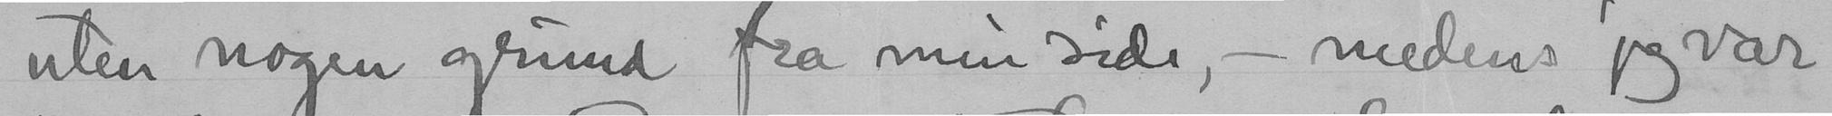uten nogen grund fra min side, - medens jeg var
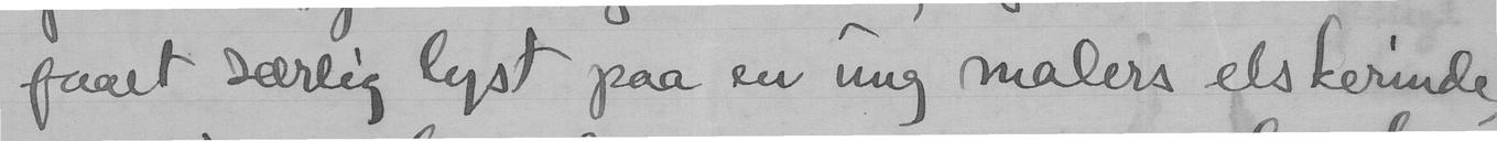faaet særlig lyst paa en ung malers elskerinde,
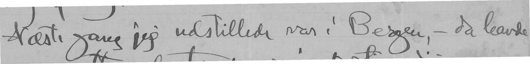Næste gang jeg udstillede var i Bergen, - da havde
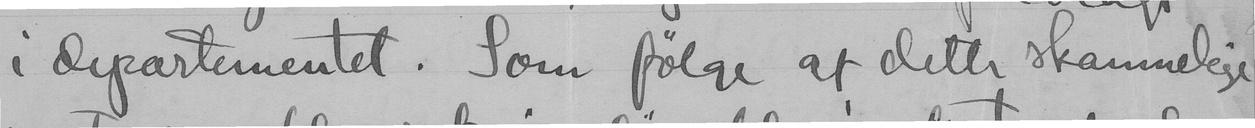i departementet. Som følge af dette skammelige
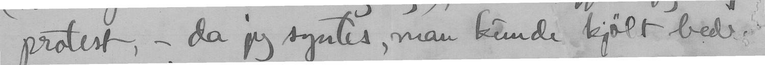protest, - da jeg syntes, man kunde kjøbt bedre
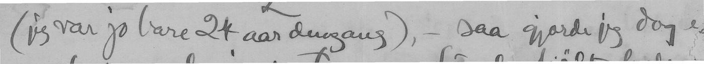(jeg var jo bare 24 aar dengang), - saa gjorde jeg dog i
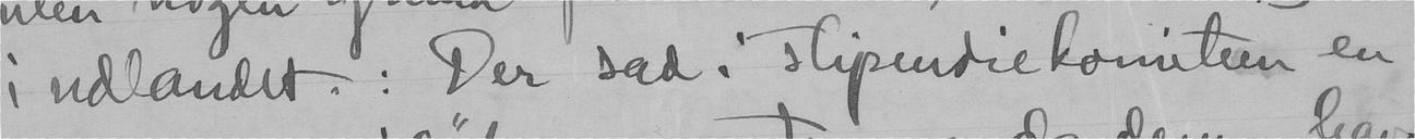i udandet: Der sad i stipendiekomiten en
